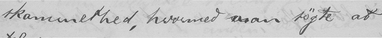skammethed, hvormed man søgte at
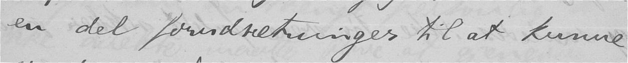en del forudsætninger til at kunne
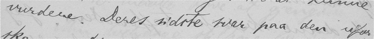vurdere. Deres sidste svar paa den ufor-
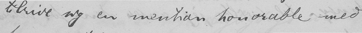tilrive sig en mention honorable med
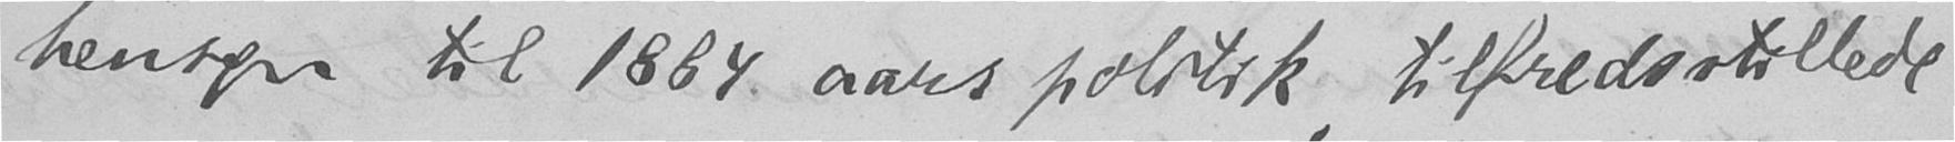hensyn til 1884 aars politik, tilfredsstillede
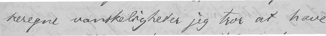særegne vanskeligheder jeg tror at have
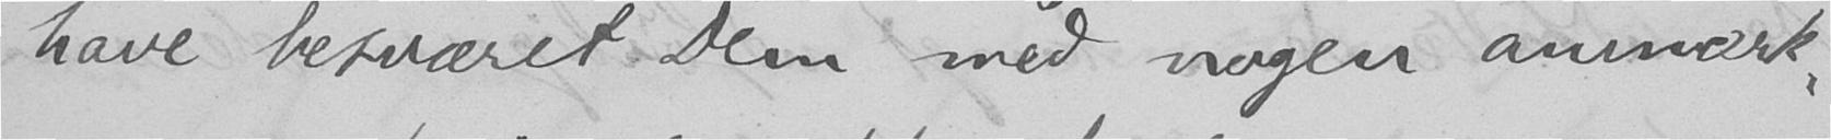have besværet Dem med nogen anmærk-
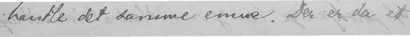handle det samme emne. Der er da et
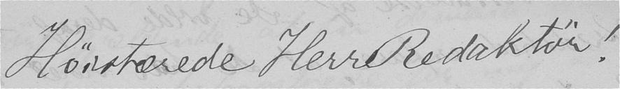Høistærede Herr Redaktør!
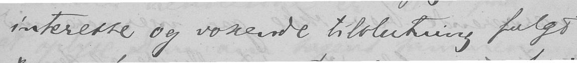interesse og voxende tilslutning fulgt
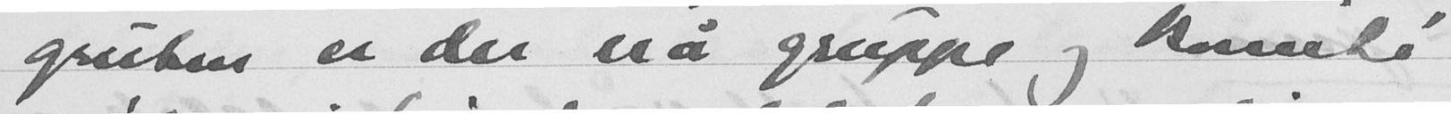gruben er det nå gruppe og komité
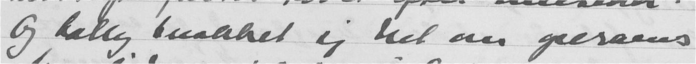Og Cally snakket sig het om operaens
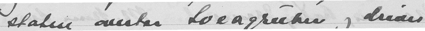staten overtar Sveagruben og driver
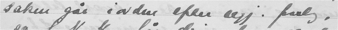saken går iorden efter regj. forslag,
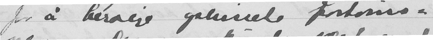for å berolige ophissede portvins-
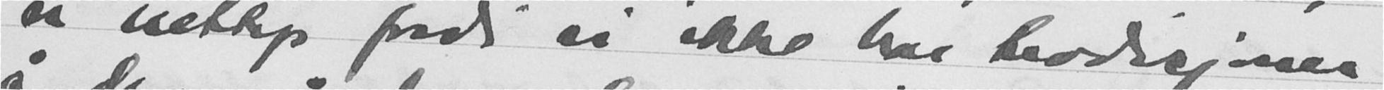vi nettop fordi vi ikke har tradisjoner
